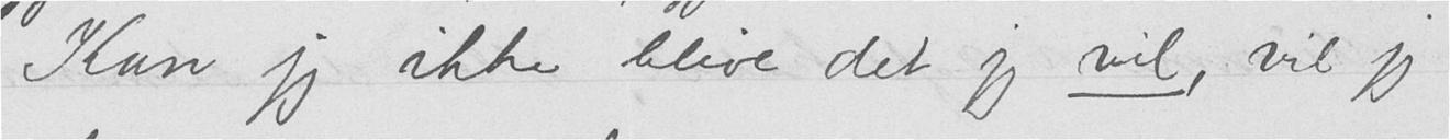Kan jeg ikke blive det jeg vil, vil jeg
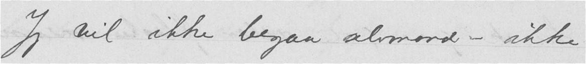Jeg vil ikke begaa selvmord - ikke
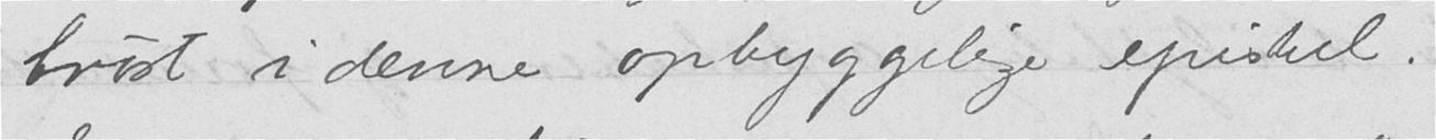trøst i denne opbyggelige epistel.
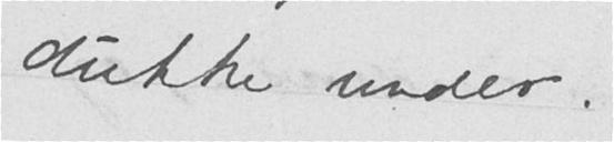dukke under.
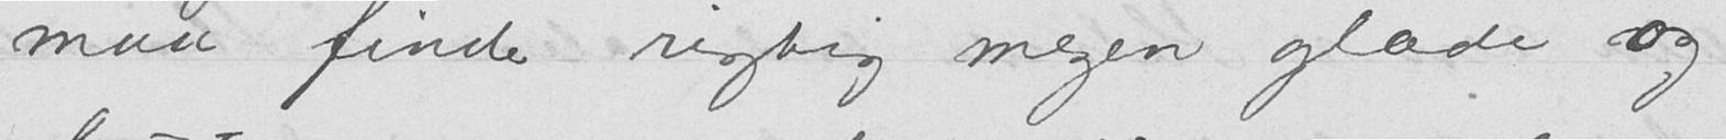maa finde rigtig megen glæde og
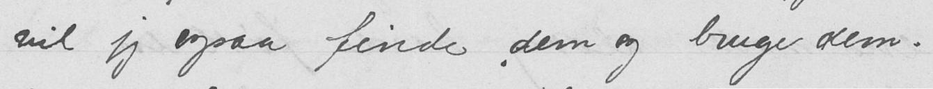vil jeg ogsaa finde dem.
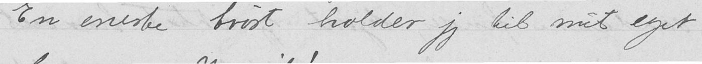En eneste trøst holder jeg til mit eget
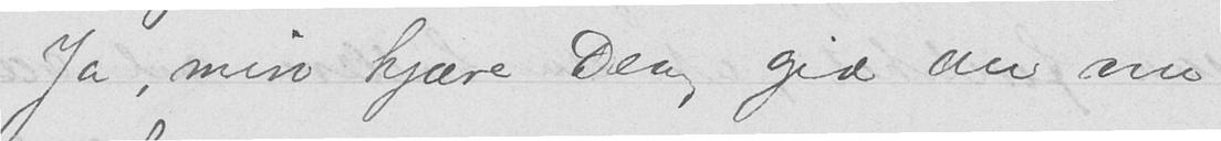Ja, min kjære Dea, gid du nu
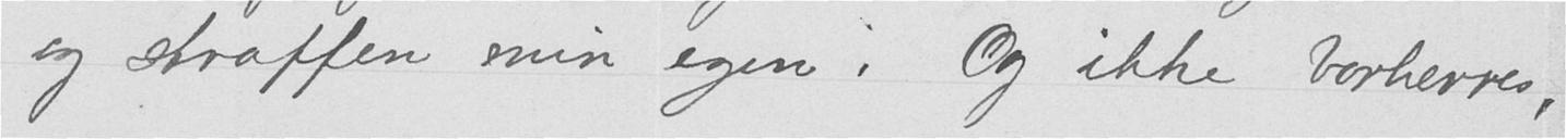og straffen min egen. Og ikke Vorherres,
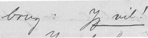brug: Jeg vil!
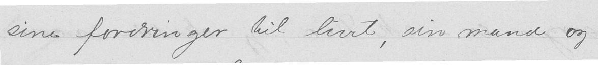sine fordringer til livet, sin mand og
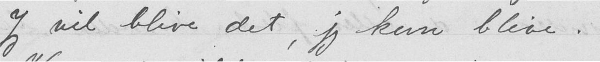Jeg vil blive det, jeg kan blive.
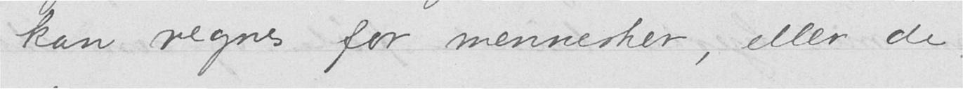kan regnes for mennesker, eller de
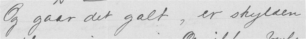Og gaar det galt, er skylden
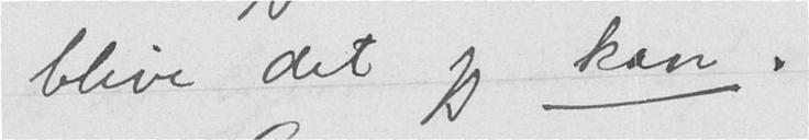blive det jeg kan.
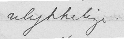ulykkelige.
